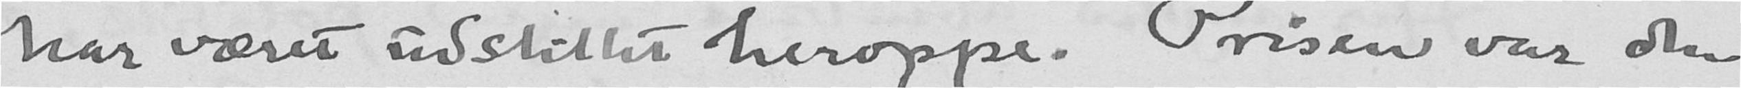har været udstillet heroppe. Prisen var den
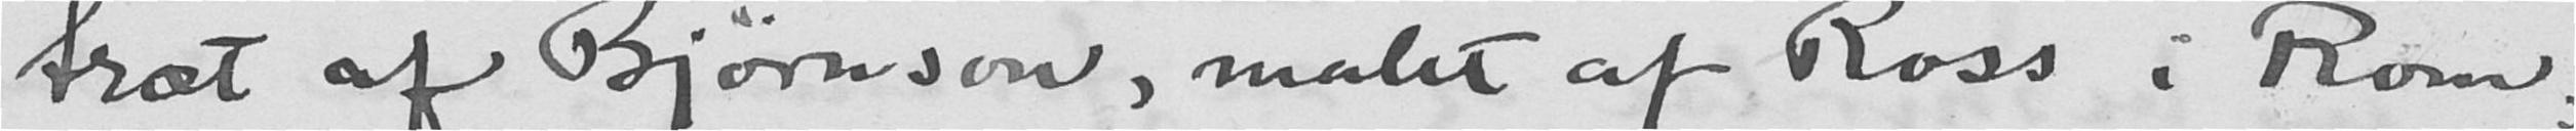træt af Bjørnson, malet af Ross i Rom,
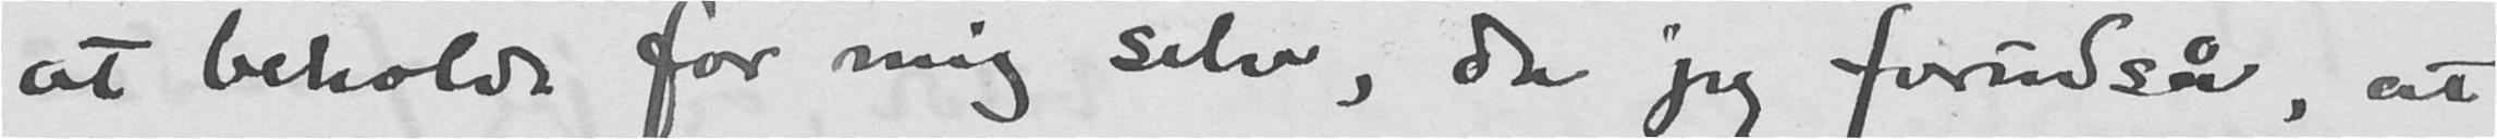at beholde for mig selv, da jeg forudså, at
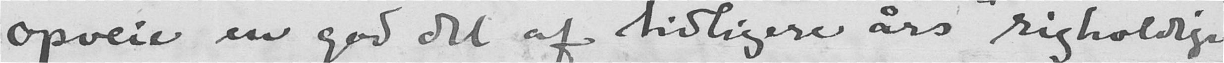opveie en god del af tidligere års "righoldige"
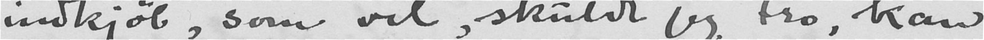indkjøb, som vel, skulde jeg tro, kan
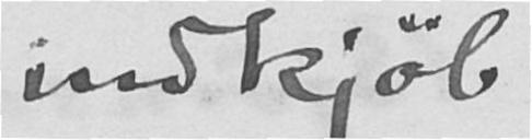indkjøb.
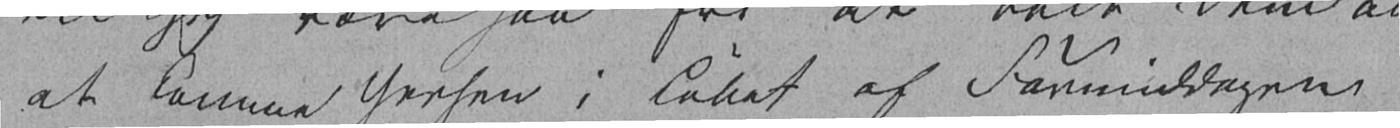at komme herhen i Løbet af Formiddagen
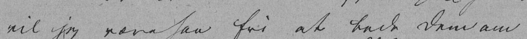vil jeg være saa fri at bede Dem om
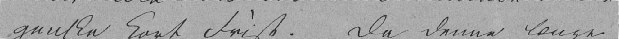ganske kort Frist. Da denne længe-
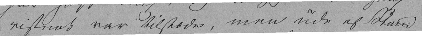vistnok var tilstæde, men ude af Stand
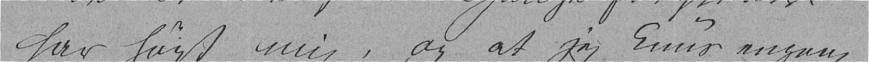har søgt mig, og at jeg kuns engang
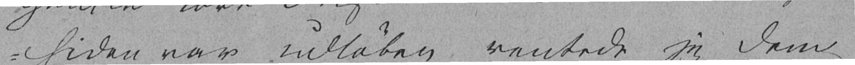siden var udløben ventede jeg Dem
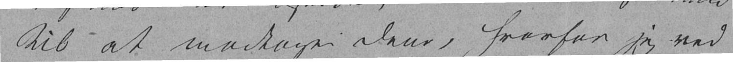til at modtage Dem, hvorfor jeg ved
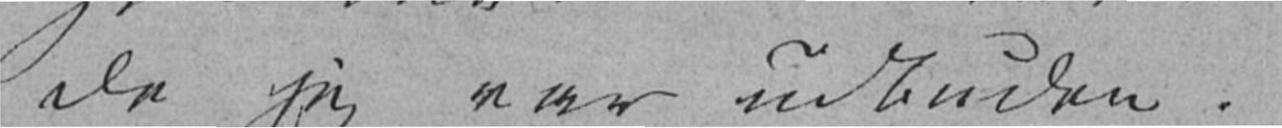da jeg var udbuden.
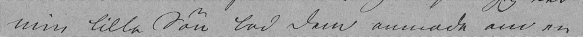min lille Søn lod Dem anmode om en
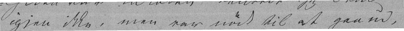igjen ikke, men var nødt til at gaa ud,
